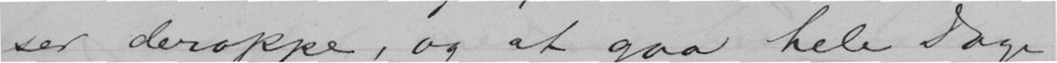ser deroppe, og at gaa hele Dage
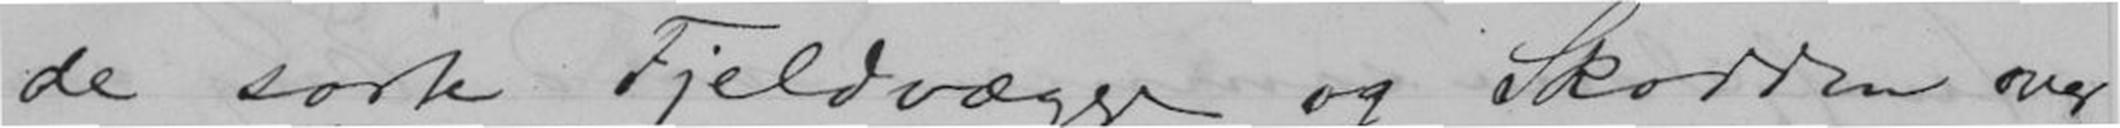de sorte Fjeldvægge og Skodden over
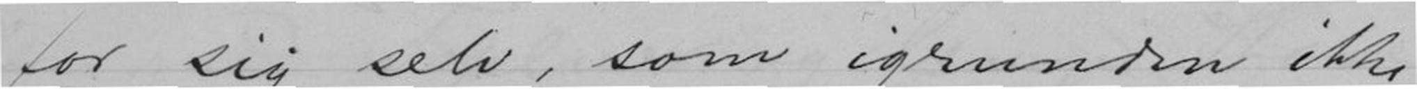for sig selv, som igrunden ikke
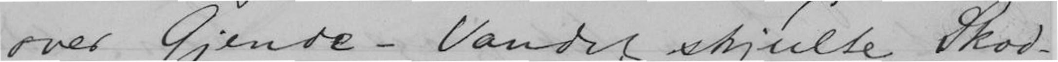over Gjende-Vandet skjulte Skod-
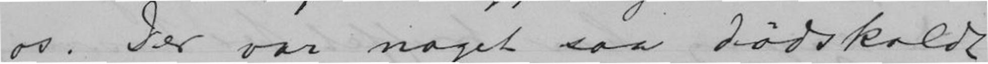os. Der var noget saa dødskoldt
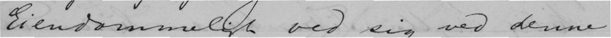Eiendommeligt ved sig ved denne
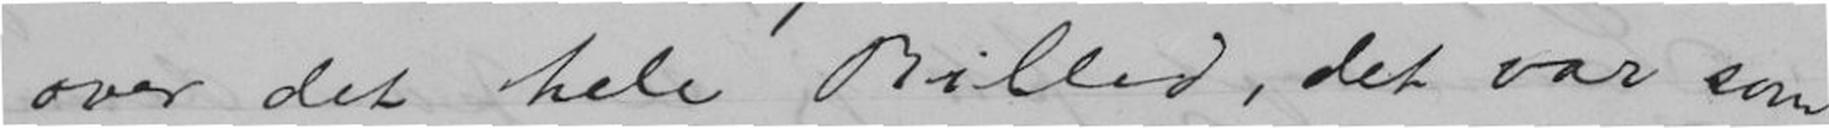over det hele Billed, det var som
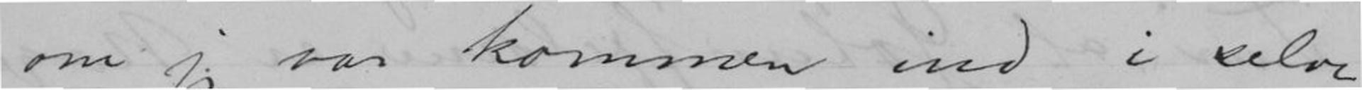om jeg var kommen ind i selve
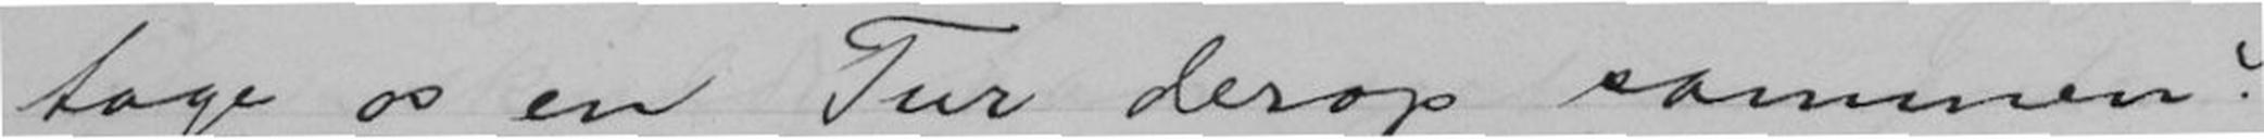tage os en Tur derop sammen.
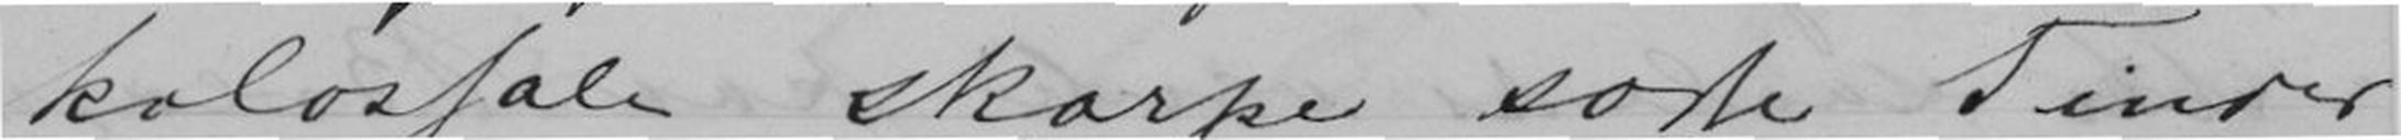kolossale skarpe sorte Tinder
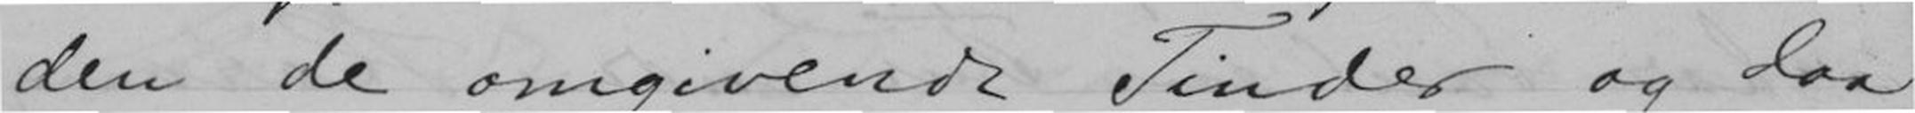den de omgivende Tinder og laa
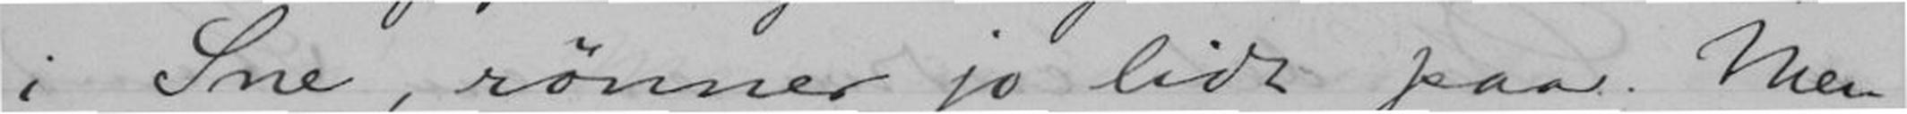i Sne, rønner jo lidt paa. Men
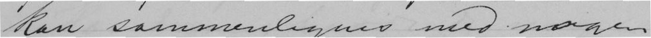kan sammenlignes med nogen
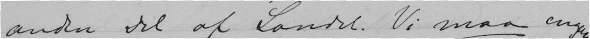anden Del af Landet. Vi maa engang
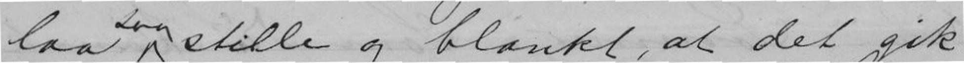laa stille og blankt, at det gik
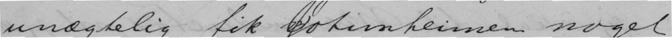unægtelig fik Jotunheimen noget
